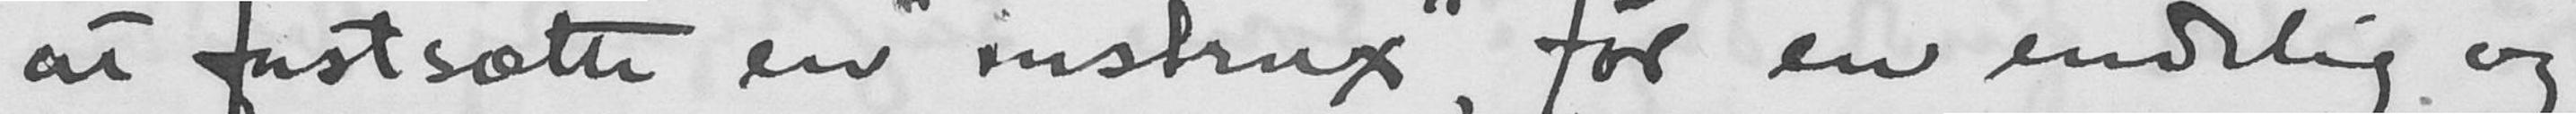at fastsætte en "instrux", før en endelig og
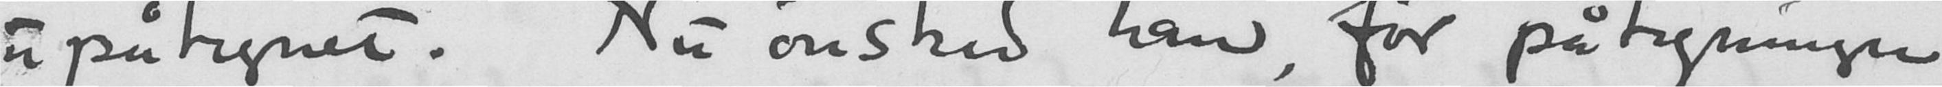upåtegnet. Nu ønsked han, før påtegningen
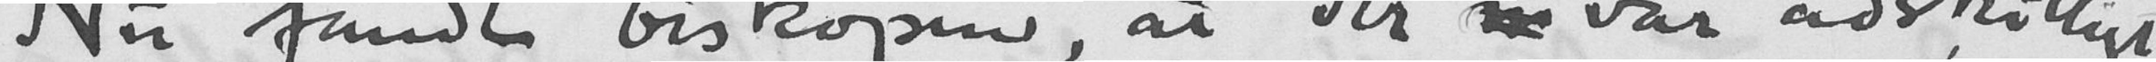Nu fandt biskopen, at der var adskilligt
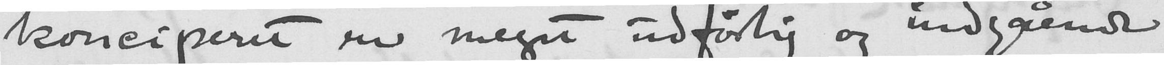konciperet en meget udførlig og indgående
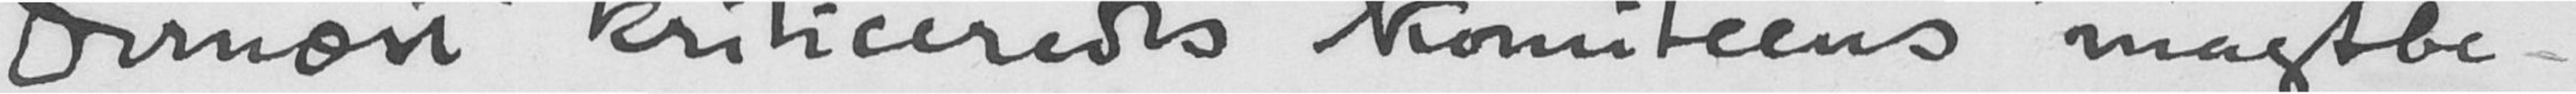Dernæst kriticeredes komiteens magtbe-
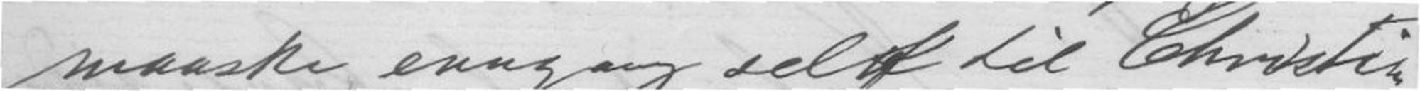maaske enngang self til Christi
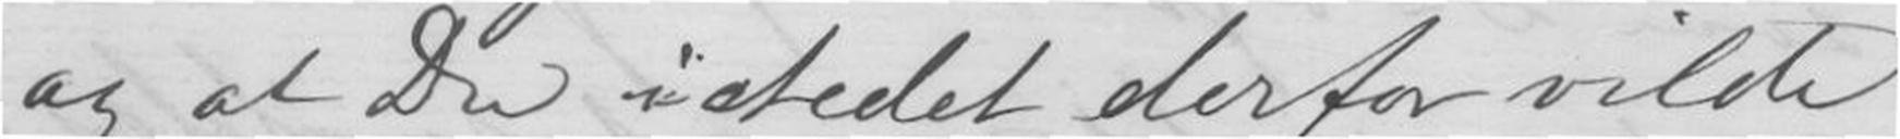og at Du istedet derfor vilde
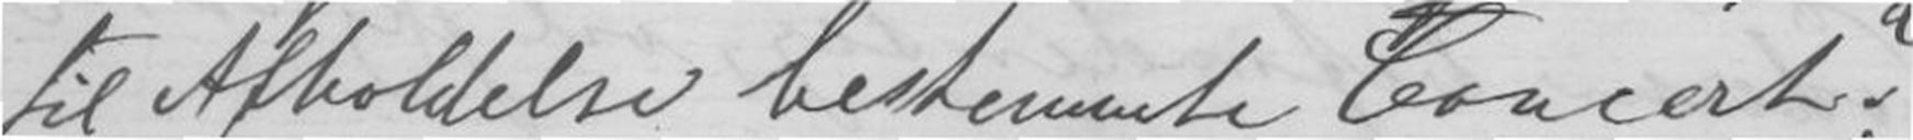til Afholdelse bestemmte Concert?
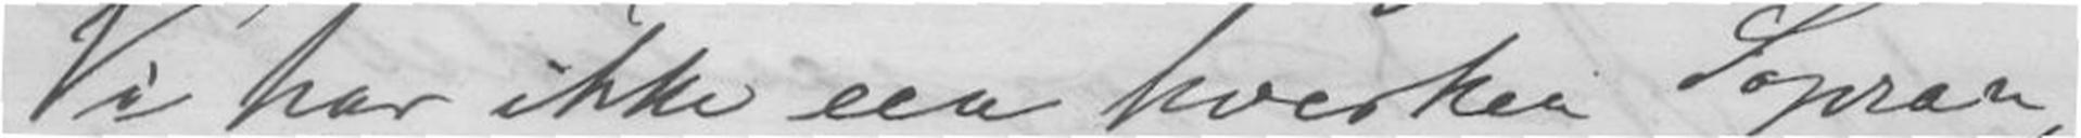Vi har ikke nu hverken Sopran,
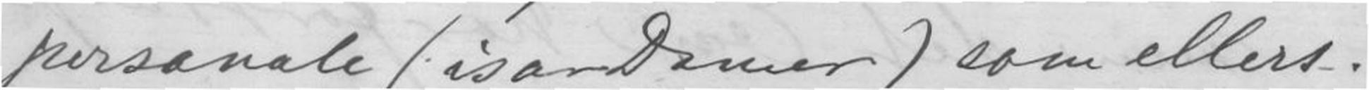personale (især Damer) som ellers.
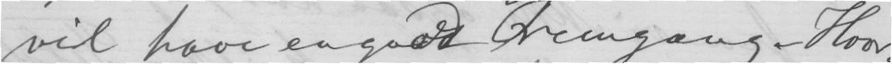vil have en good Fremgang. Hvor-
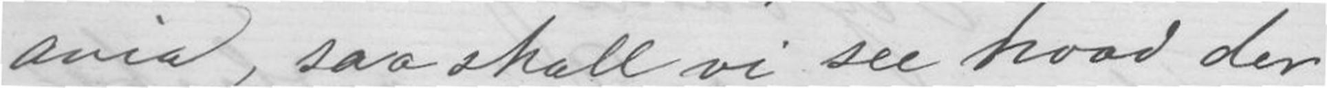ania, saa skall vi see hvad der
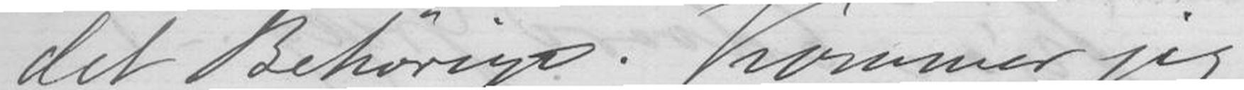det Behørige. Kommer jeg
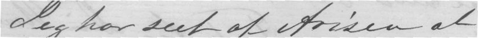Jeg har seet af Aviserne at
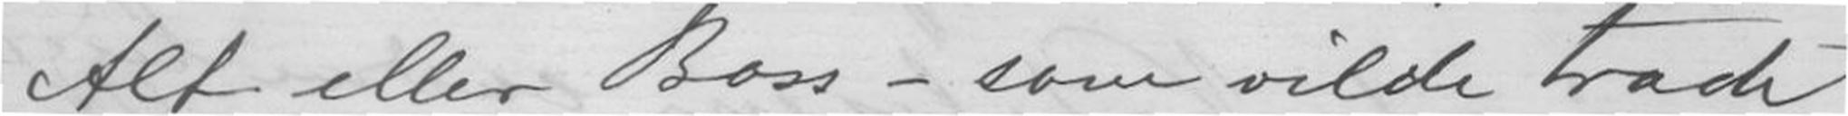Alt eller Bass - som vilde træde
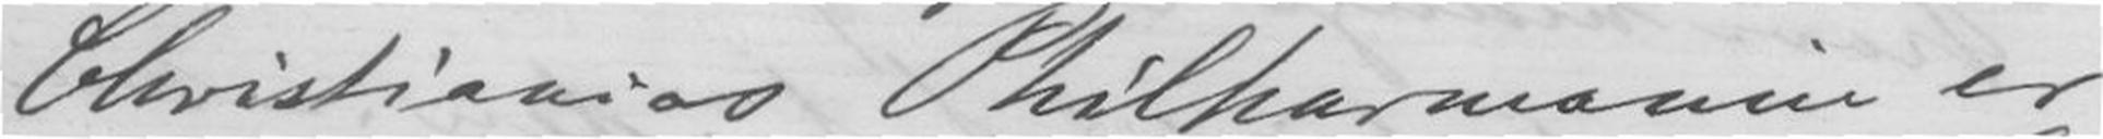Christianias Philharmonin er
